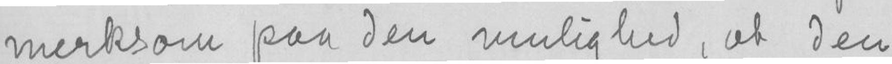mærksom paa den mulighed, at den
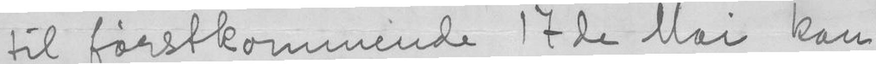til førstkommende 17de Mai kan
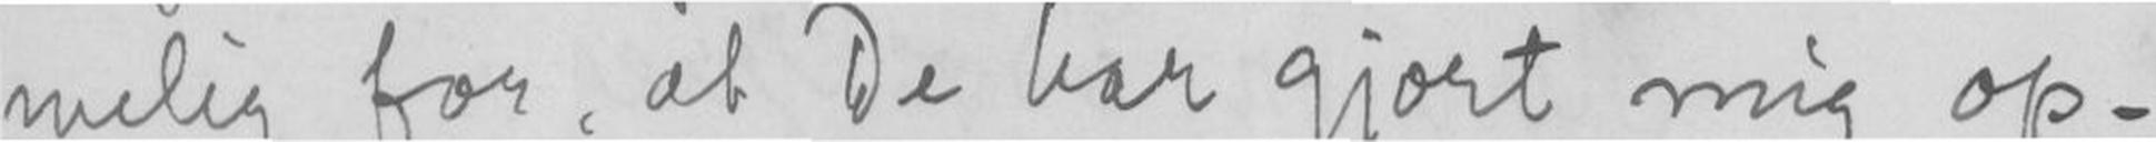melig for, at De har gjort mig op-
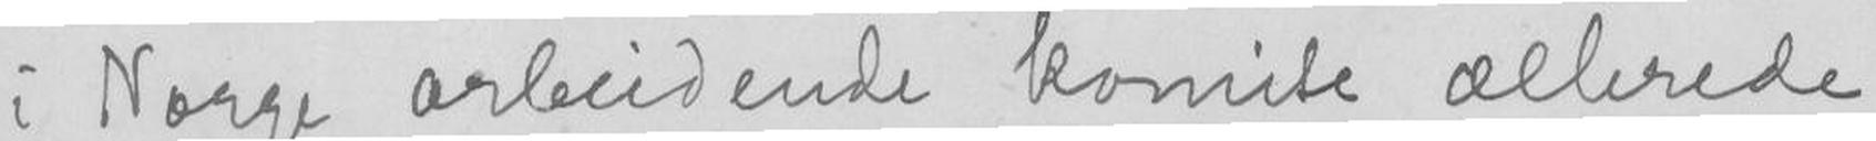i Norge arbeidende komite allerede
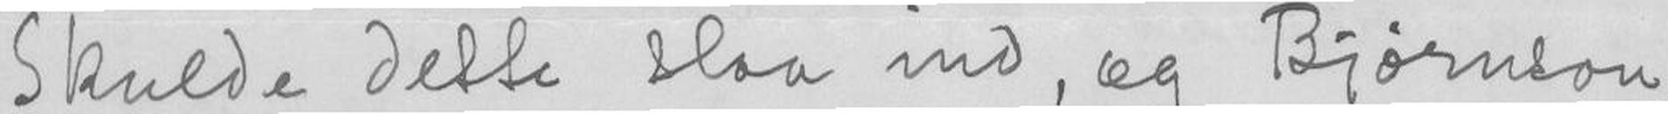Skulde dette slaa ind, og Bjørnsom
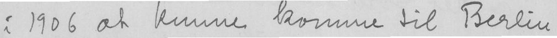i 1906 at kunne komme til Berlin,
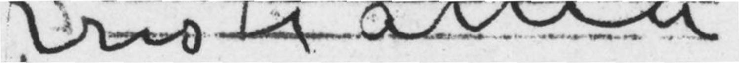Kristiania
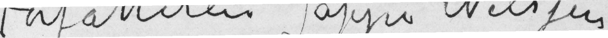Forfatteren Jappe Nilssen
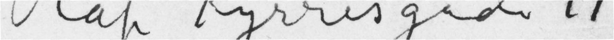Olaf Kyrres gade 11
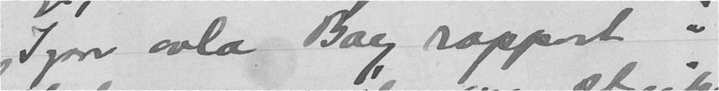Igaar avla Bay rapport i
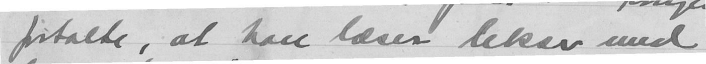fortalte, at han læser lekser med
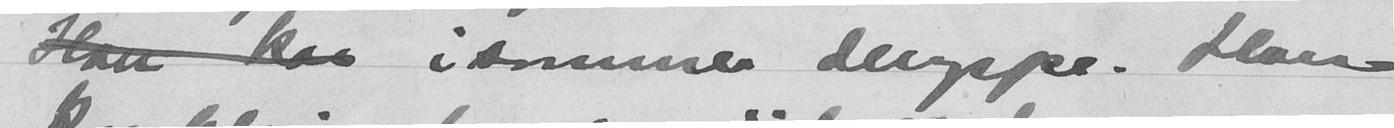Han kom i sommer deroppe. Han
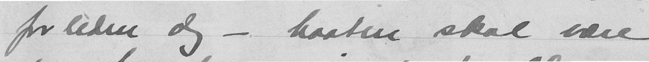forleden dag - baaten skal være
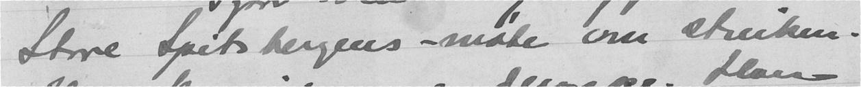Store Spitsbergens-møte om streiken.
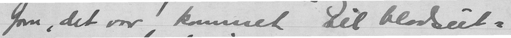paa, det var kommet til blodsut-
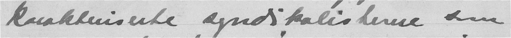karakteriserte syndikalisterne som
